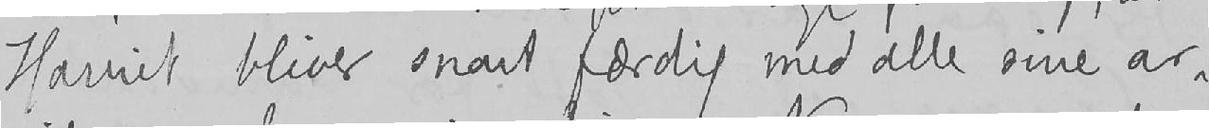Harriet bliver snart færdig med alle sine ar-
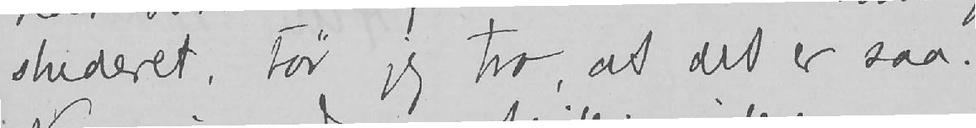studeret, tør jeg tro, at det er saa.
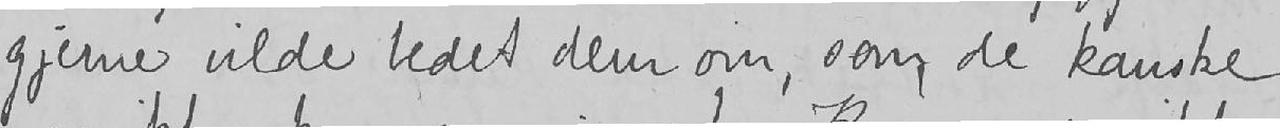gjerne vilde bedet dem om, som de kanske
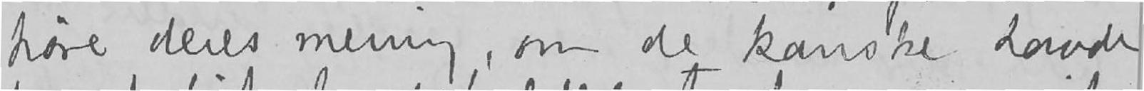høre deres mening, om de kanske havde
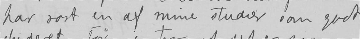har rost en af mine studier som godt
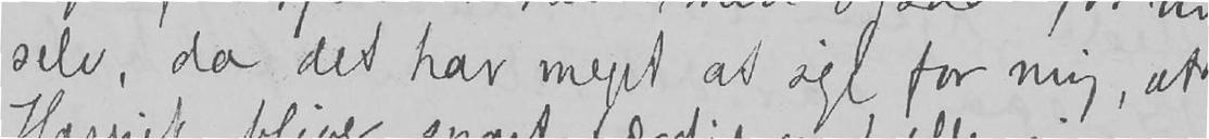selv, da det har meget at sige for mig, at
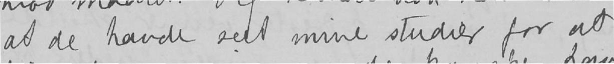at de havde seet mine studier for at
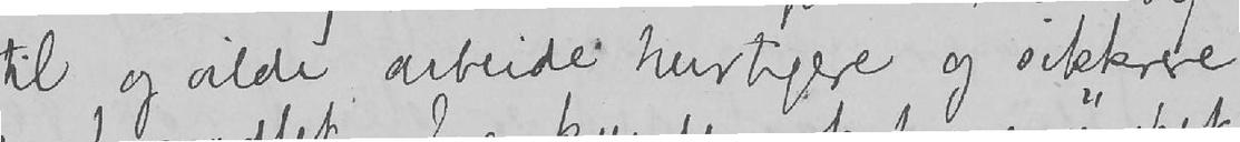til og vilde arbeide hurtigere og sikkrere
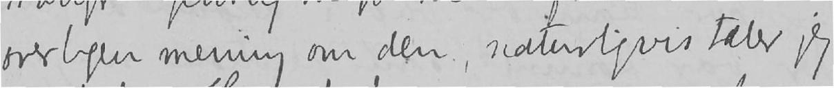overlegen mening om den, naturligvis taler jeg
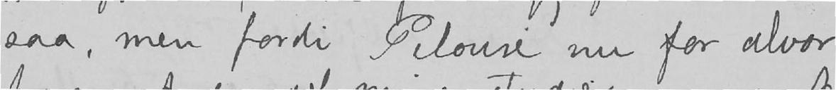saa, men fordi Pelouse nu for alvor
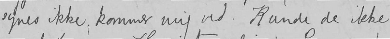synes ikke, kommer mig ved. Kunde de ikke
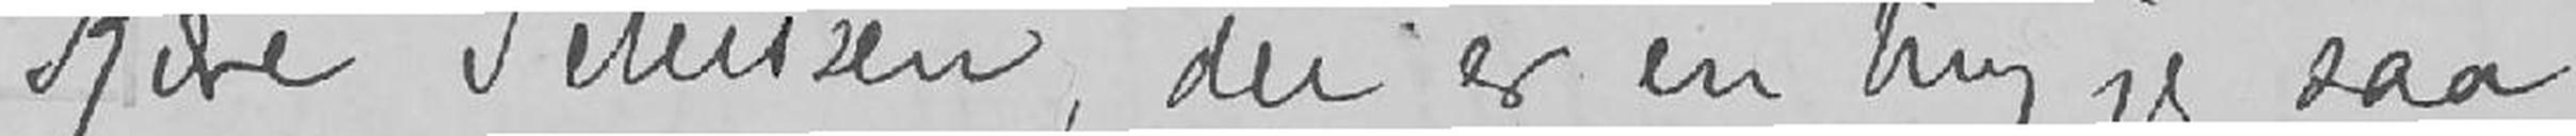Kjære Peterssen, der er en ting jeg saa
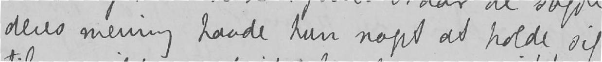deres mening havde hun noget at holde sig
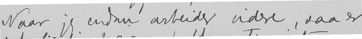Naar jeg endnu arbeider videre, saa er
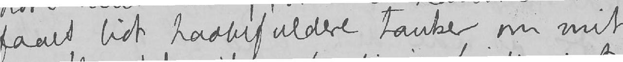faaet lidt haabefuldere tanker om mit
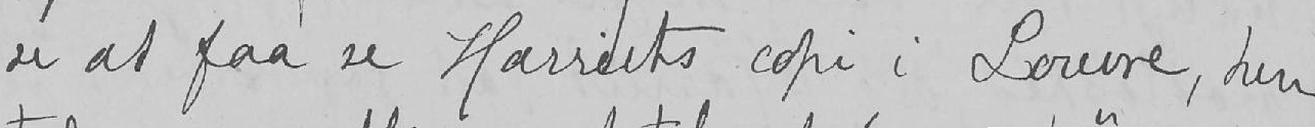se at faa se Harriets copi i Louvre, hun
